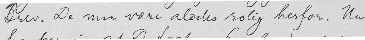Brev. De maa være aldeles rolig herfor. Nu
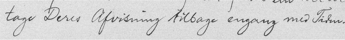tage Deres Afvisning tilbage engang med Tiden.
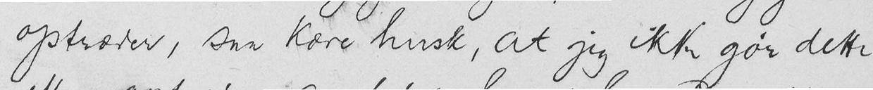optræder, saa kære husk, at jeg ikke gør dette
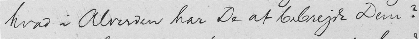hvad i alverden har De at bebrejde Dem?
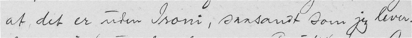at det er uden Ironi, saasandt som jeg lever.
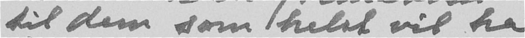til dem som helst vil ha
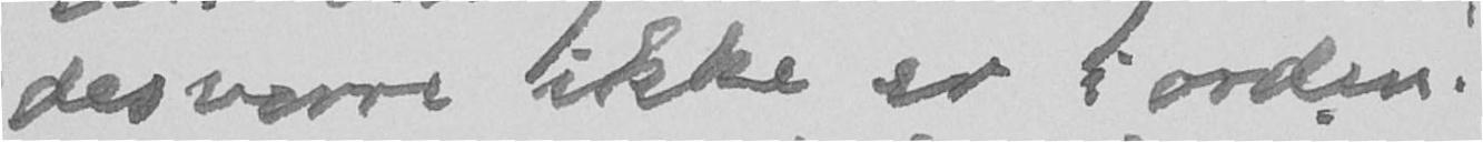desverre ikke er i orden!
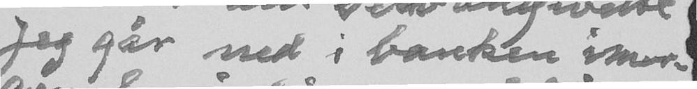Jeg går ned i banken imor-
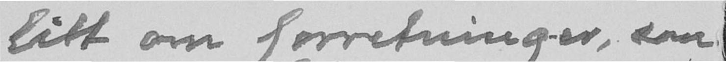litt om forretninger, som
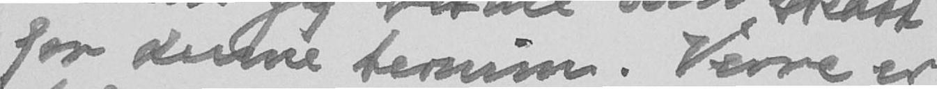for denne termin. Verre er
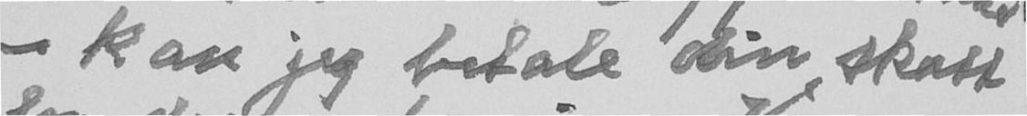- kan jeg betale din skatt
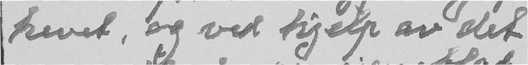hevet, og ved hjelp av det
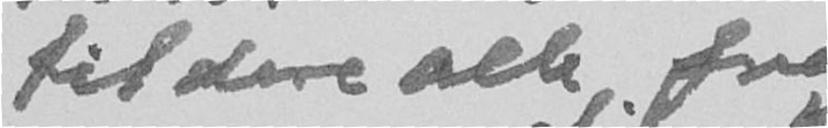til dere alle fra
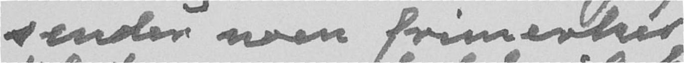sender noen frimerker
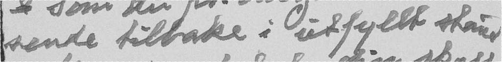sende tilbake i utfyllt stånd
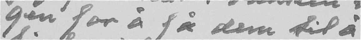gen for å få dem til å
